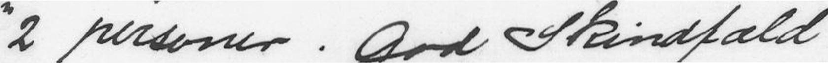"2 personer. God Skindfæld"
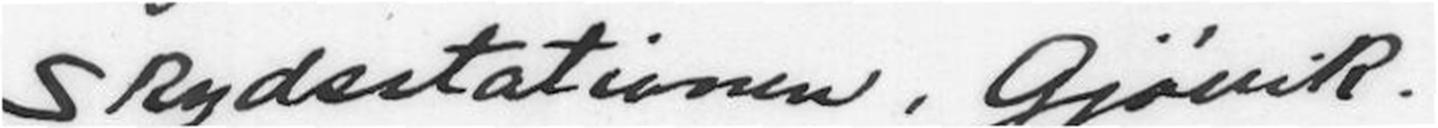Skydstationen, Gjøvik.
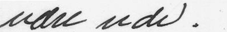være ude.
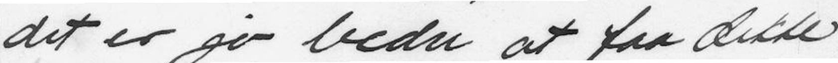det er jo bedre at faa dette
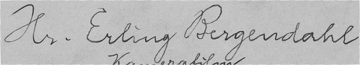Hr. Erling Bergendahl
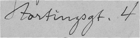Stortingsgt. 4
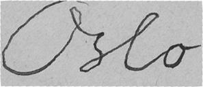Oslo
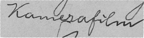Kamerafilm
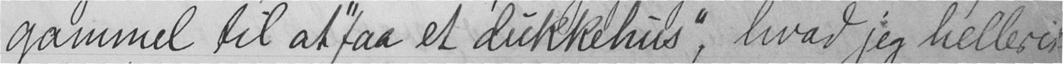gammel til at "faa et dukkehus", hvad jeg hellerikke
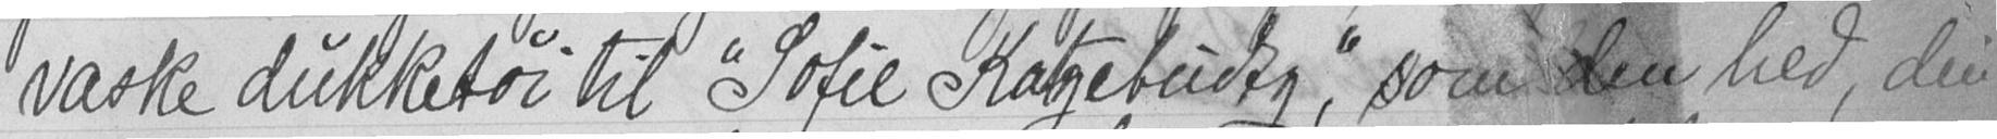vaske dukketøi til "Sofie Kagebudtg," som den hed, den
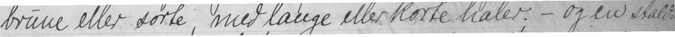brune eller sorte, med lange eller korte haler; - og en stald-
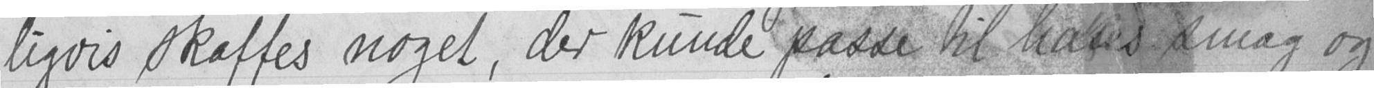ligvis skaffes noget, der kunde passe til hans smag og
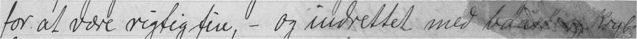for at være rigtig fin, - og indrettet med baade
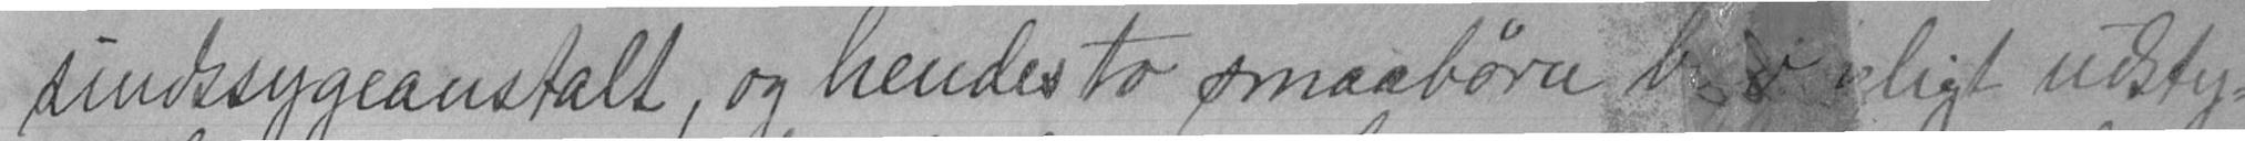sindssygeanstalt, og hendes to smaabørn blir uligt udsty-
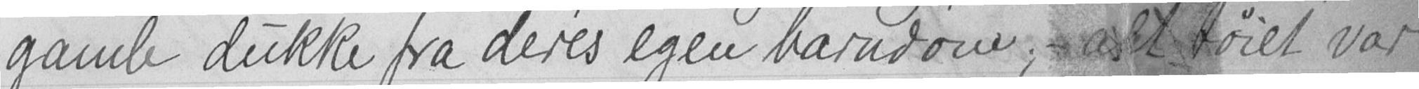gamle dukke fra deres egen barndom; alt tøiet var
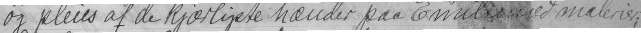og pleies af de kjærligste hænder paa Emilsminde med malerier;
